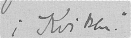i Kirken."
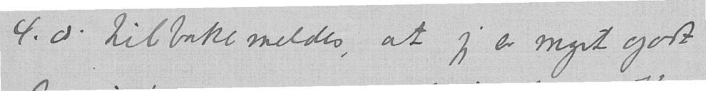4. ds. tilbakemeldes, at jeg er meget godt
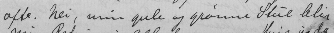ofte. Nei, min gule og grønne stue blir
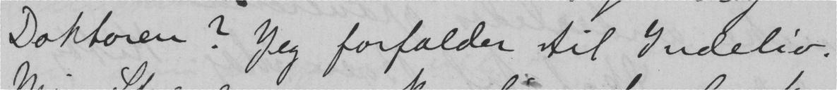Doktoren? Jeg forfalder til Indeliv.
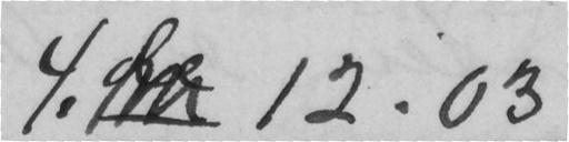4.12.03
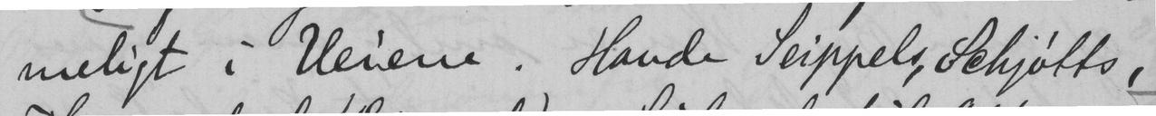meligt i veiene. Havde Seippels Schjøtts,
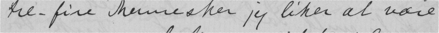tre-fire mennesker jeg liker at være
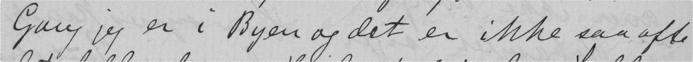Gang jeg er i Byen og det er ikke saa ofte
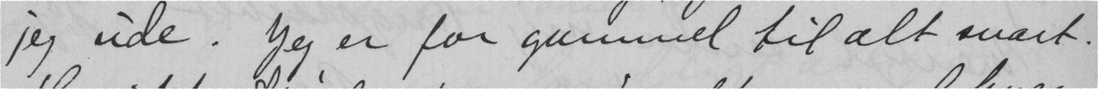jeg ude. Jeg er for gammel til alt svært.
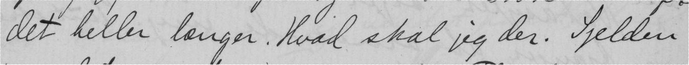det heller længer. Hvad skal jeg der. Sjelden
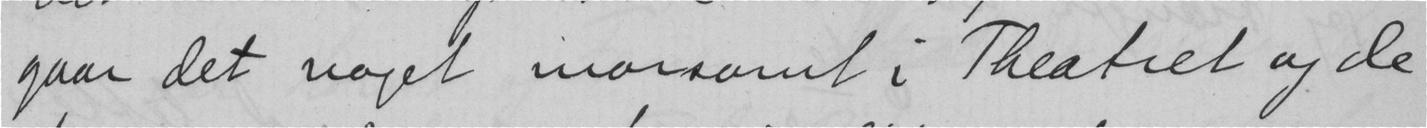gaar det noget morsamt i Theatret og de
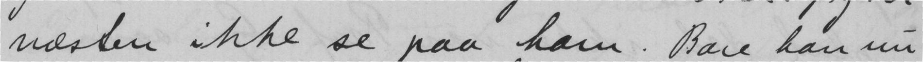næsten ikke se paa ham. Bare han nu
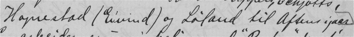Hognestad (Eivind) og Løland til aftens igaar
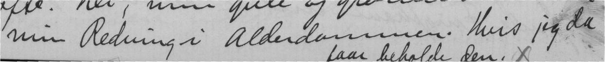min Redning i alderdommen. Hvis jeg da
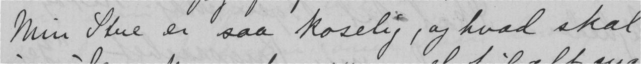Min Stue er saa koselig, og hvad skal
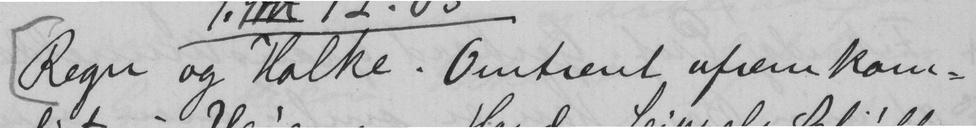Regn og Holke. Omtrent ufremkom-
